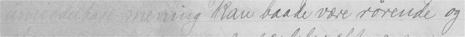umiddelbare mening kan baade være rørende og
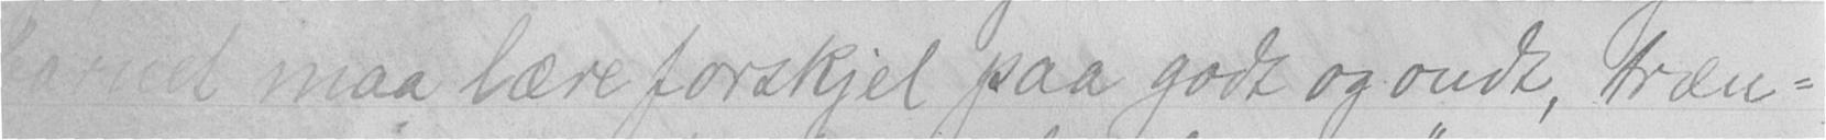barnet maa lære forskjel paa godt og ondt, træn-
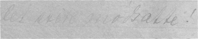det stik modsatte!
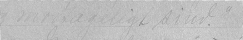og modtageligt sind."
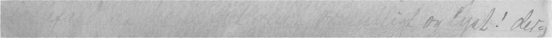og lyst! der-
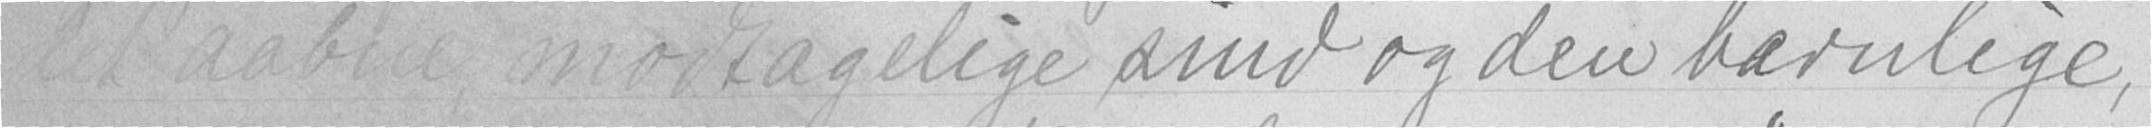det aabne, modtagelige sind og den barnlige,
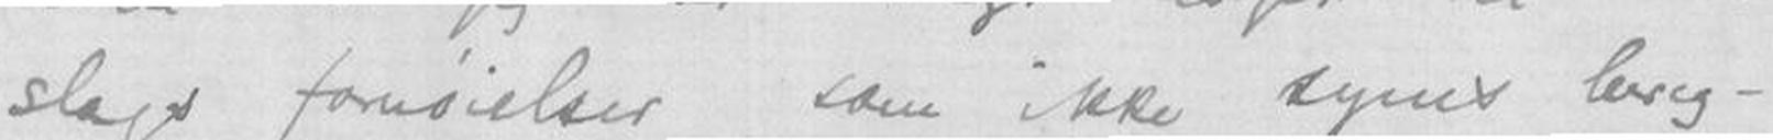slags fornøielser, som ikke syntes bereg-
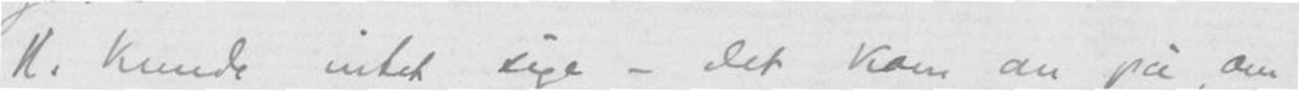H. kunde intet sige - det kom an på, om
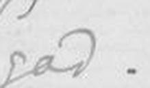god.
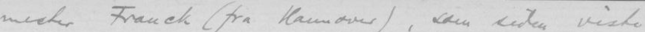mester Franck (fra Hannover), som siden viste
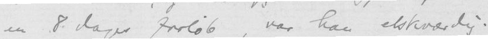en 8 dages forløb, var han elskværdig-
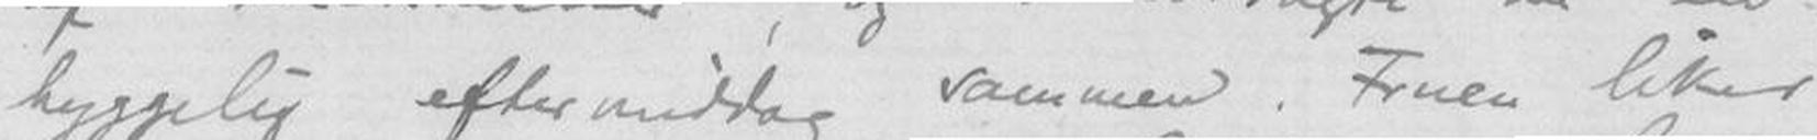hyggelig eftermiddag sammen. Fruen liker
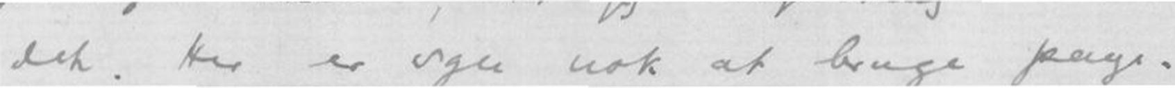det. Her er sgu nok at bruge penge-
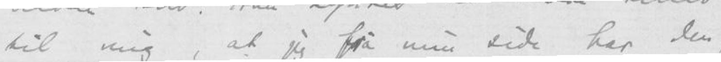til mig, at jeg frå min side har den,
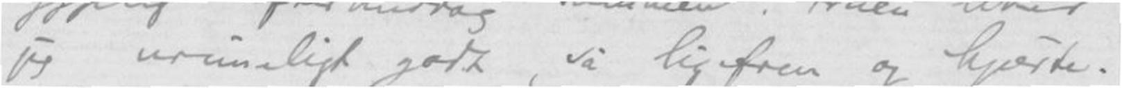jeg urimeligt godt, så ligefrem og hjerte-
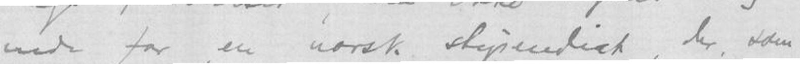nede for en norsk stipendiat, der som
